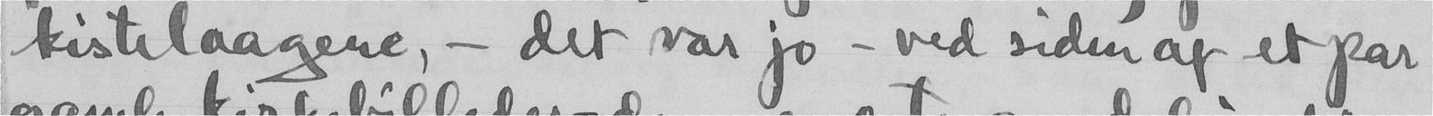kistelaagene, - det var jo - ved siden af et par
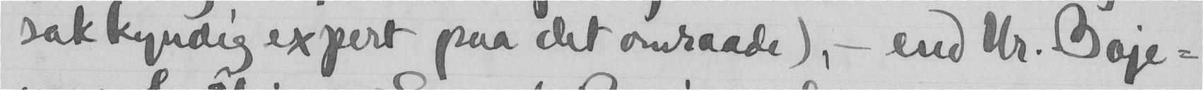sakkyndig expert paa det omraade), - end Hr. Boje-
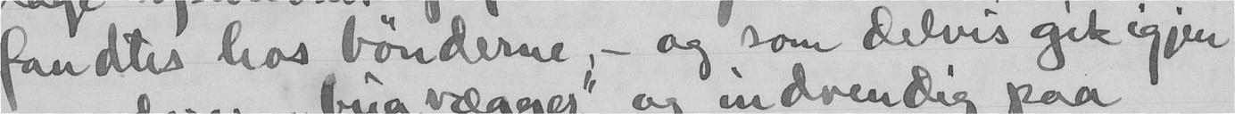fandtes hos bønderne, - og som delvis gik igjen
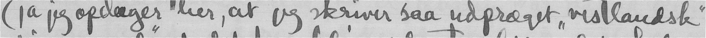(ja jeg opdager her, at jeg skriver saa udpræget "vestlandsk"
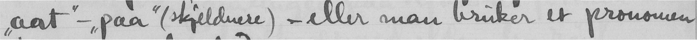"aat" - "paa" (skjeldnere) - eller man bruker et pronomen
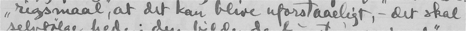"rigsmaal", at det kan blive uforstaaeligt, - det skal
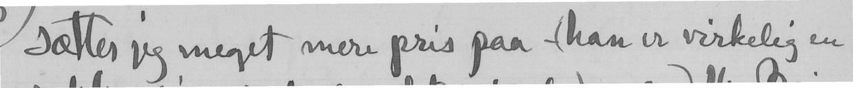Sætter jeg meget mere pris paa - (han er virkelig en
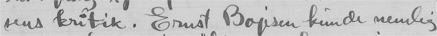sens krikik. Ernst Bojesen kunde nemlig
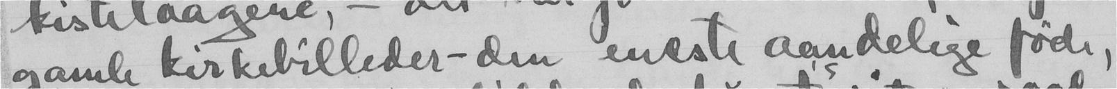gamle kirkebilleder - den eneste aandelige føde,
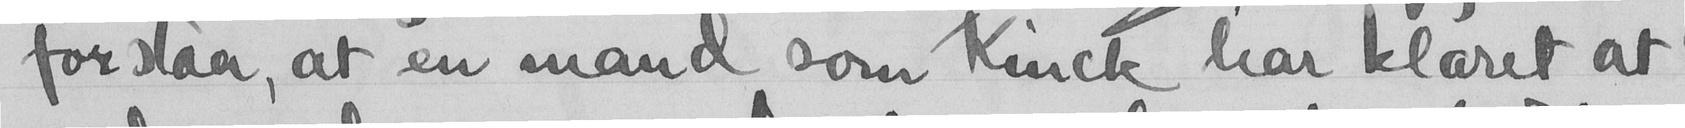forstaa, at en mand som Kinck har klaret at
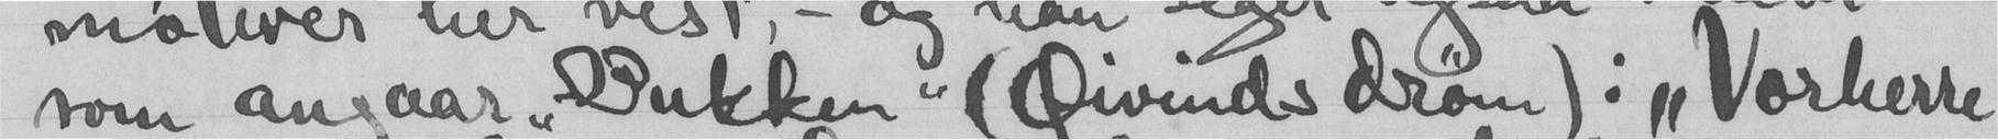som angaar "Bukken" (Øivinds drøm): "Vorherre
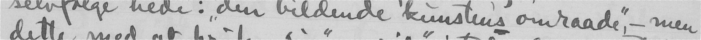selvfølge hede: "den bildende kunstens omraade", - men
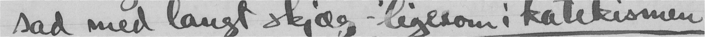sad med langt skjæg - ligesom i katekismen
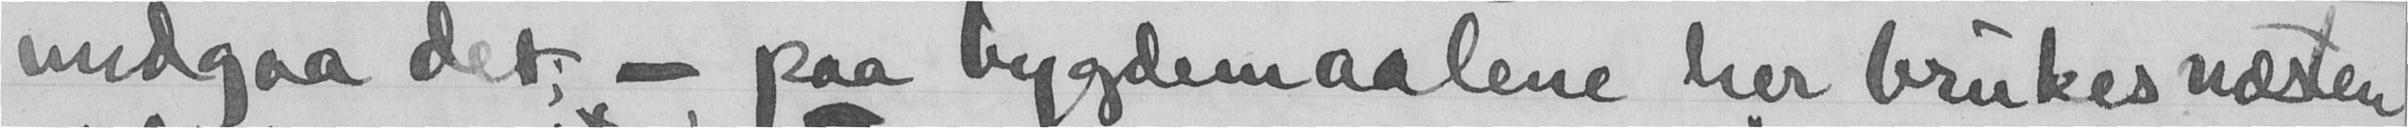undgaa det, - paa bygdemaalene her brukes næsten
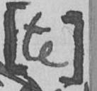(te)
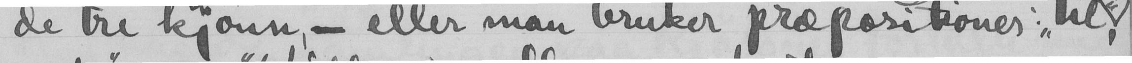de tre kjønn, - eller man bruker præpositioner: "til"
